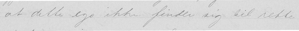at dette ego ikke finder sig til rette
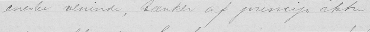eneste veninde, tænker af princip ikke
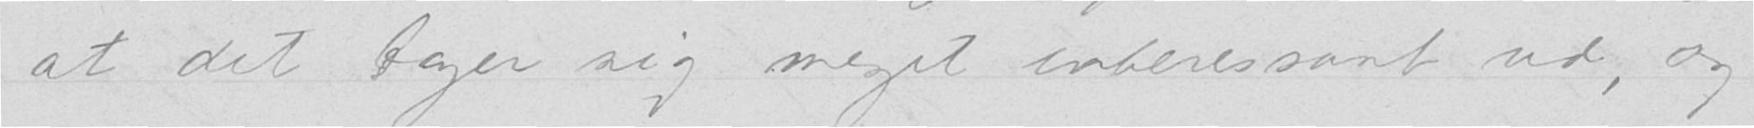at det tager sig meget interessant ud, og
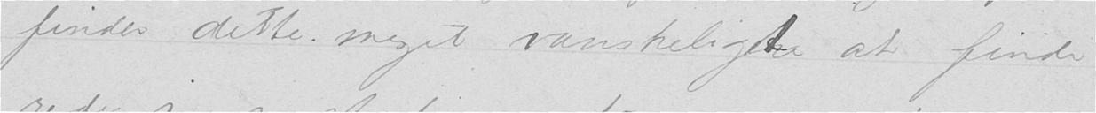finder dette meget vanskeligt at finde
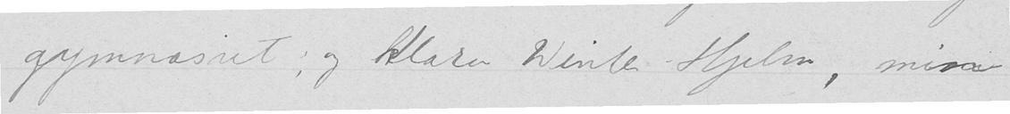gymnasiet; og Klara Winter-Hjelm, min
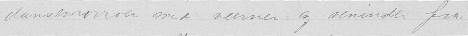dansemorroer med venner og veninder fra
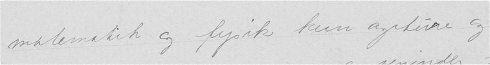matematikk og fysik kun ageture og
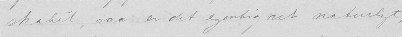skabet, saa er det egentlig ret naturligt,
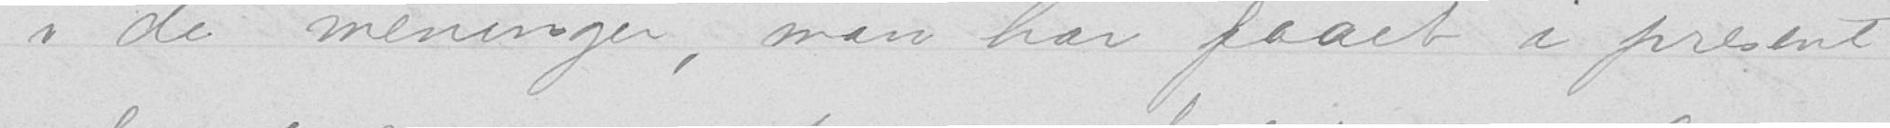i de meninger, man har faaet i present
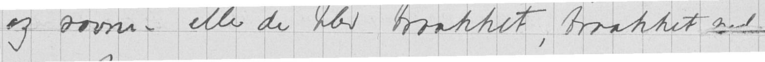og savne – eller de blev traakket, traakket ned -.
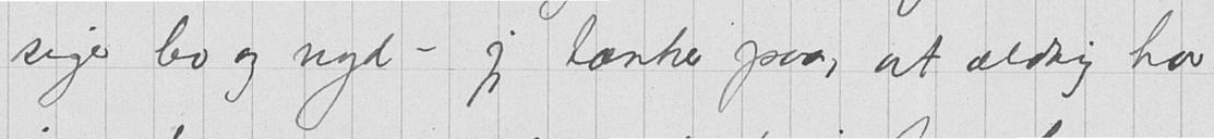siger lev og nyd – jeg tænker paa, at aldrig har
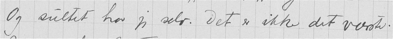Og sultet har jeg selv. Det er ikke det værste.
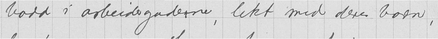bodd i arbeidergaderne, lekt med deres børn,
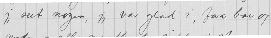jeg seet nogen, jeg var glad i, fara leve og
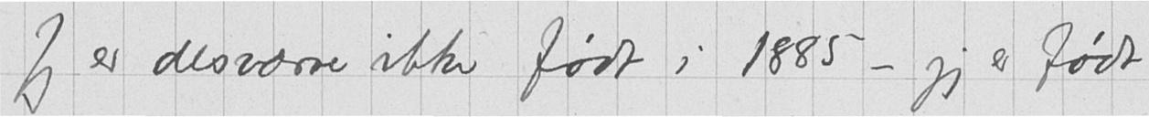Jeg er desværre ikke født i 1885 — jeg er født
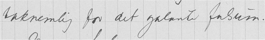taknemlig for det galante falsum.
